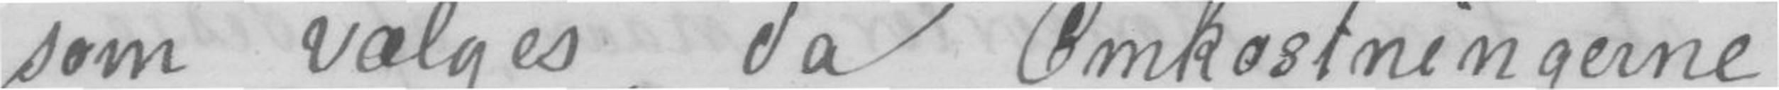som vælges, da Omkostningerne
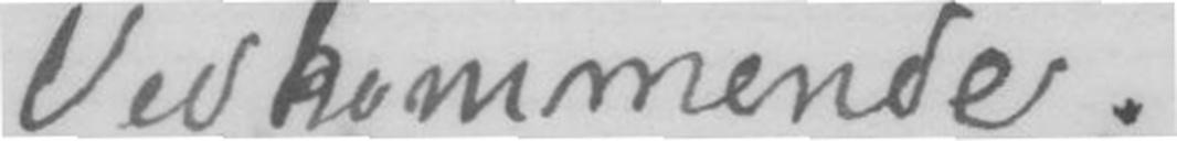Velkommende.
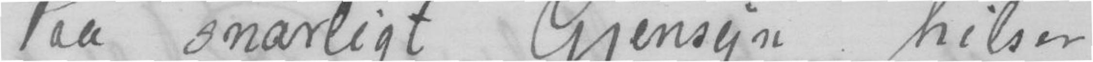Paa snarligt Gjensyn hilsen
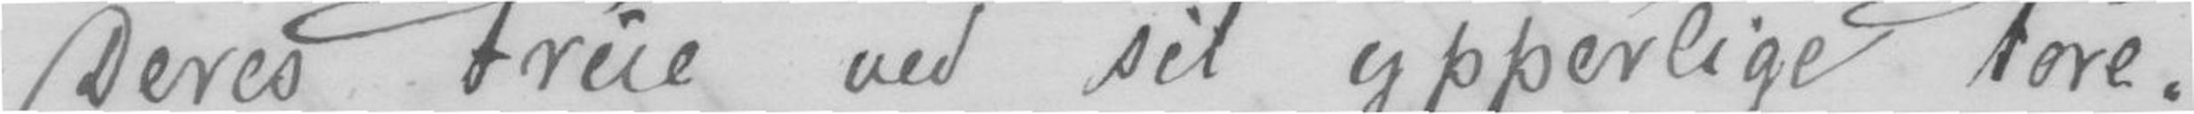Deres Frue ved sit ypperlige Fore-
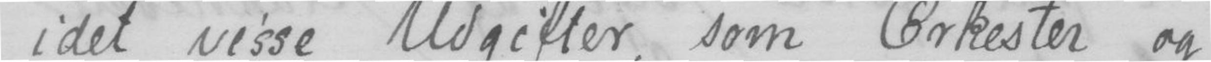idet visse Udgifter, som Orkester og
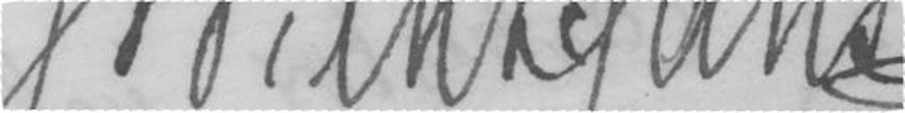Wilhelm Hans
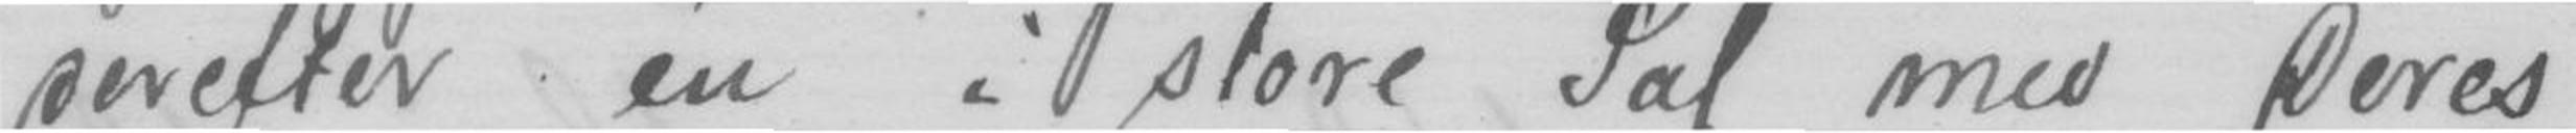serefter en i store Sal med Deres
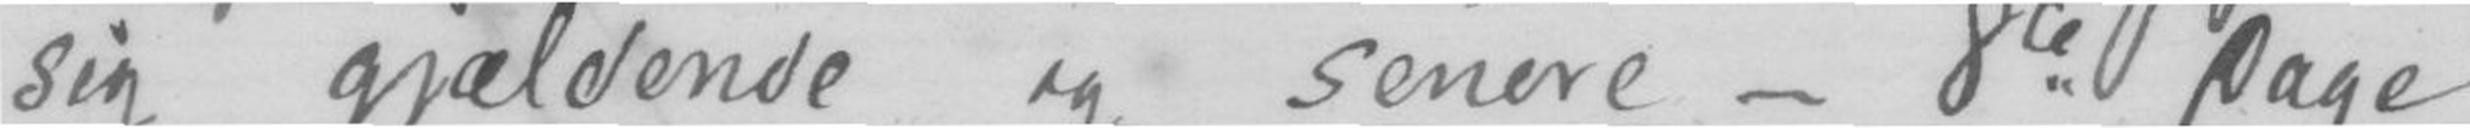sig gjældende og senere - 8te Dage
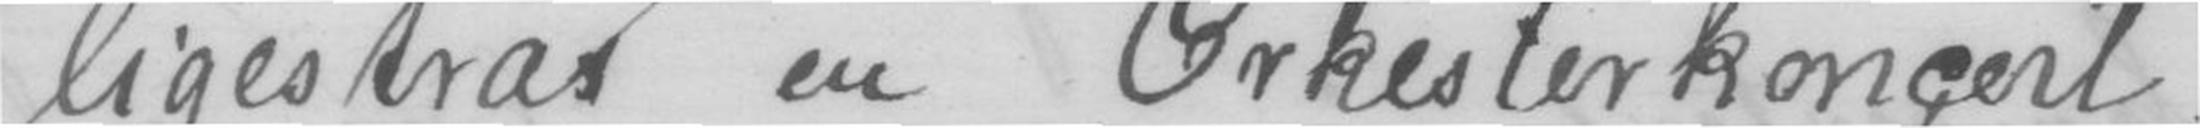ligestras en Orkesterkoncert.
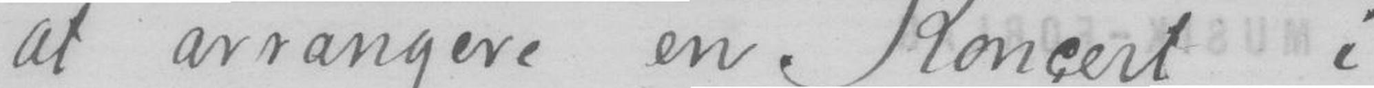at arrangere en Koncert i
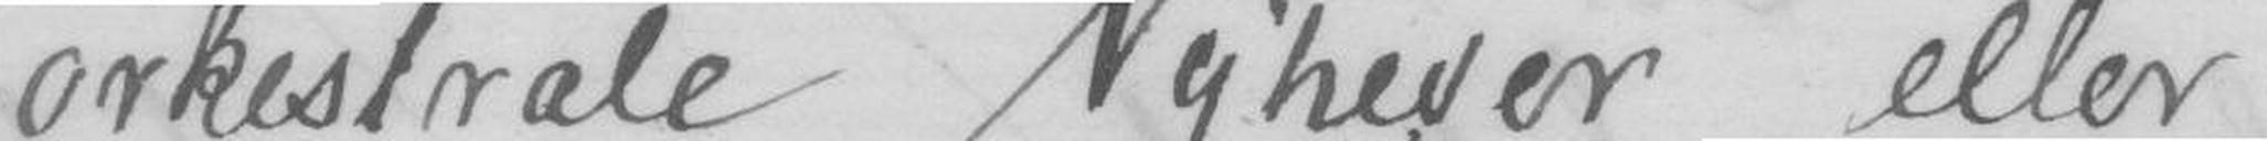orkestrale Nyheder eller
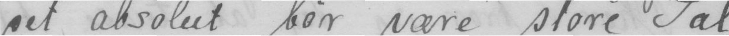Det absolut bør være store Sal
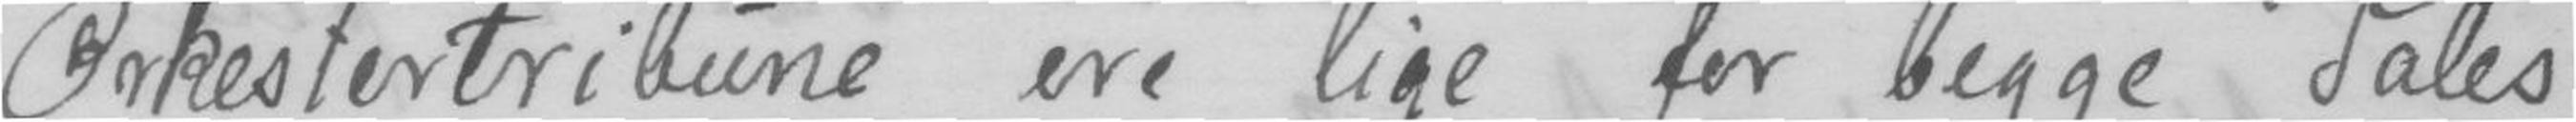Orkestertribune ere lige for begge Sales
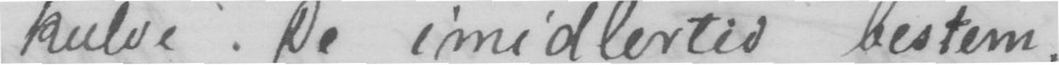Skulde De imidlertid bestem
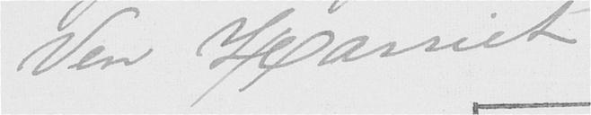Ven Harriet
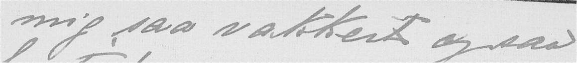mig saa vakkert og saa
